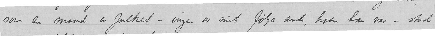som en mand av folket – ingen av mit følge ante, hvem han var - stod
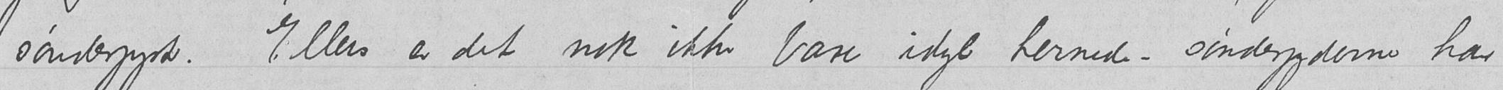sønderjyst. Ellers er det nok ikke bare idyl hernede – sønderjyderne har
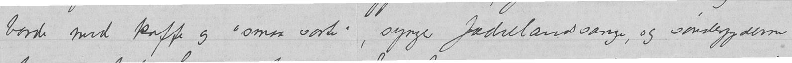borde med kaffe og "smaa sorte", synger fædselandssange, og sønderjyderne
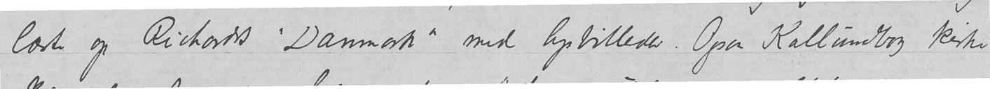læste op Richards "Danmark" med lysbilleder. Ogsaa Kallundborg kirke
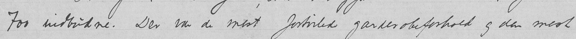for indbudne. Der var de mest fortvilede garderobeforhold og den mest
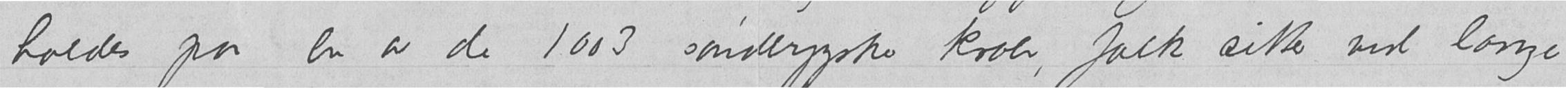holdes paa en av de 1003 sønderjyske kroer, folk sitter ved lange
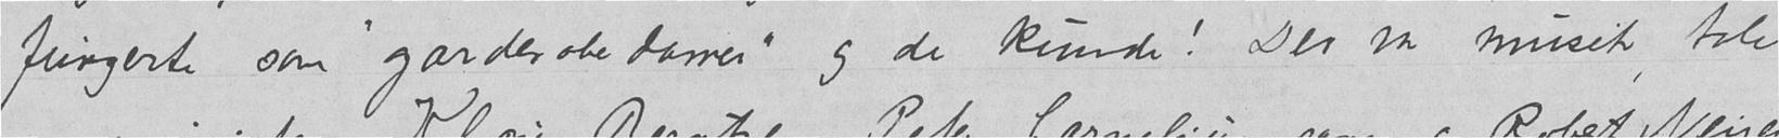fungerte som "garderobedamer" og de kunde! Der var musik, taler
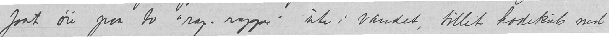faat øie paa to "rap-rapper" ute i vandet, trillet hodekuls ned
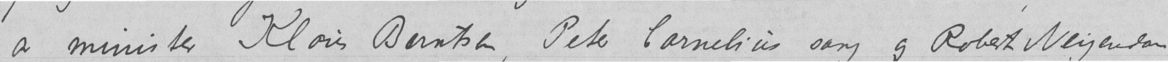av minister Klaus Berntsen, Peter Cornelius sang og Robert Neijendam
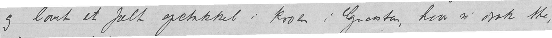og lavet et fælt spetakkel i kroen i Graasten, hvor vi drak the,
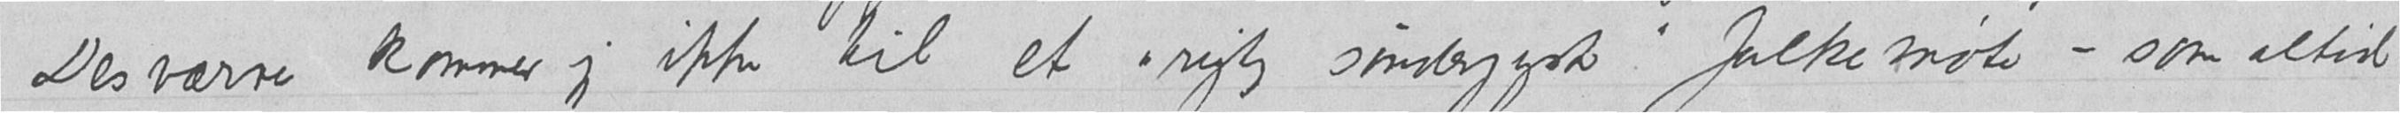Desværre kommer jeg ikke til et "rigtig sønderjysk" folkemøte – som altid
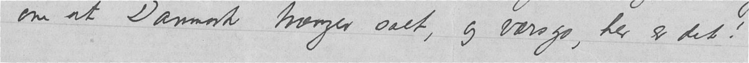om at Danmark trænger salt, og værsgo, her er det!
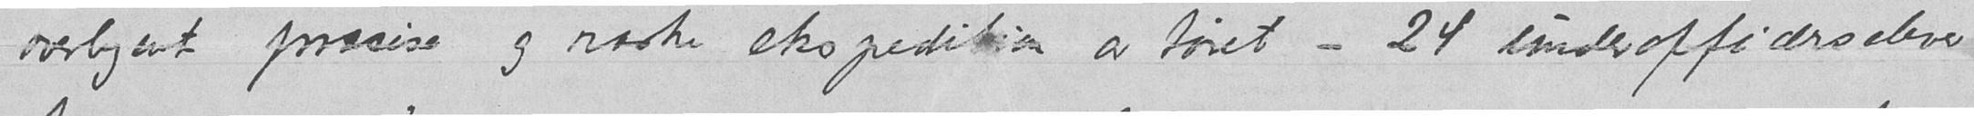overlegent præcise og raske ekspedition av tøiet – 24 underofficærselever
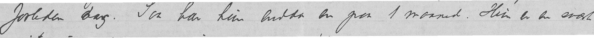forleden dag. Saa har hun endda en paa 1 maaned. Hun er en svært
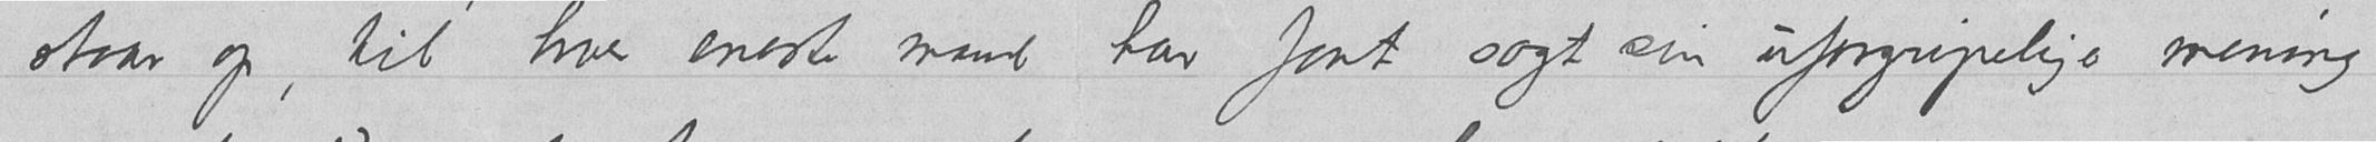staar op, til hver eneste mand har faat sagt sin uforgripelige mening
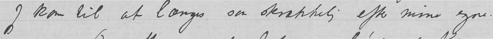Jeg kom til at længes saa skrækkelig efter mine egne.
